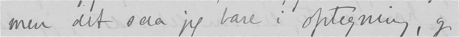men det saa jeg bare i optegning, og
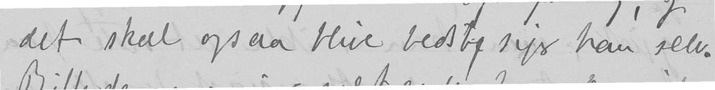det skal ogsaa blive bedst, siger han selv.
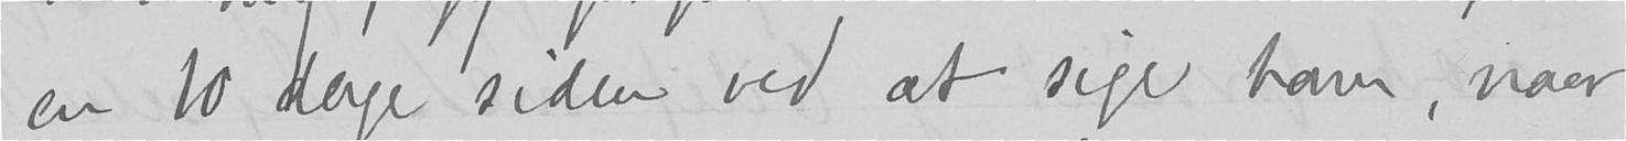en 10 dage siden ved at sige ham, naar
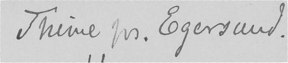Thime pr. Egersund.
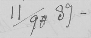11/90 89.
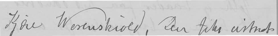Kjære Werenskiold. Du fik vistnok
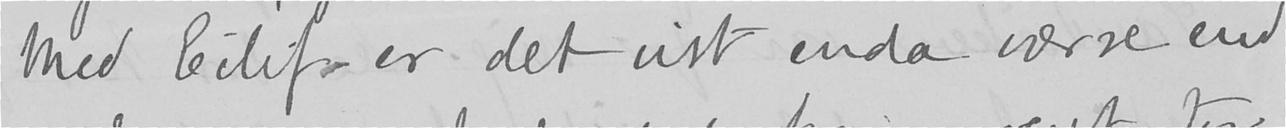Med Eilif er det vist enda værre end
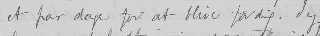et par dage for at blive færdig. Jeg
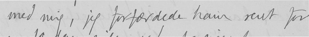med mig, jeg forfærdede ham rent for
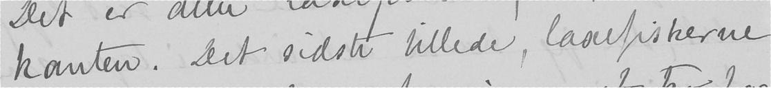kanten. Det sidste billede, laxefiskerne
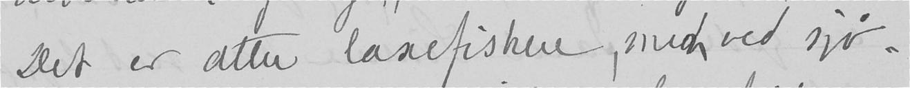Det er atter laxefiskere, ned ved sjø-
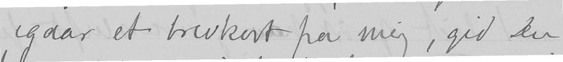igaar et brevkort fra mig, gid Du
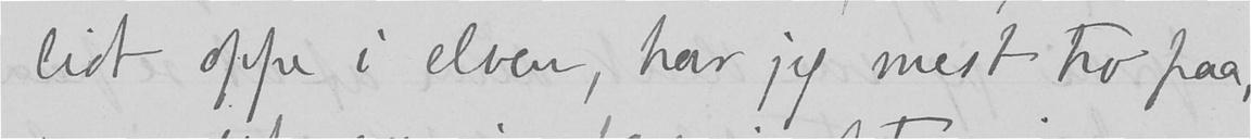lidt oppe i elven, har jeg mest tro paa,
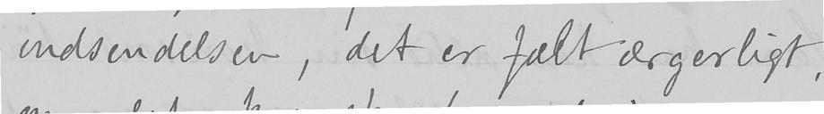indsendelsen, det er fælt ærgerligt,
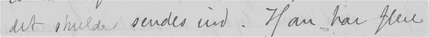det skulde sendes ind. Han har flere
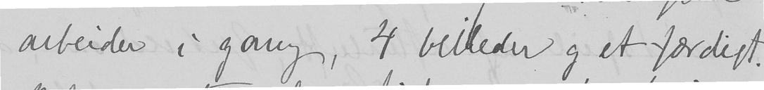arbeider i gang, 4 billeder og et færdigt.
